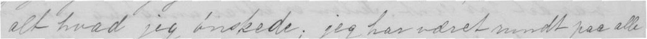alt hvad jeg ønskede; jeg har været rundt paa alle
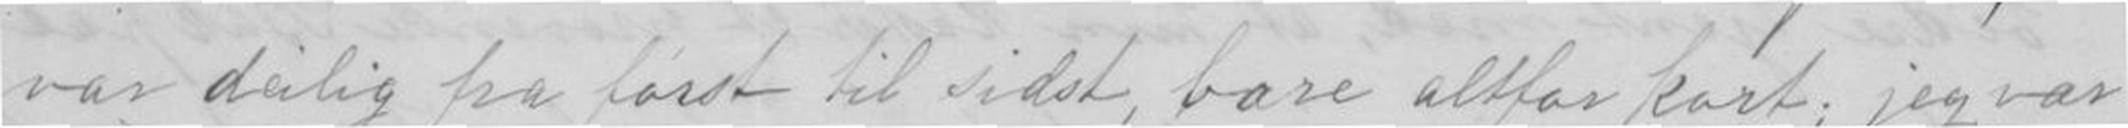var deilig fra først til sidst, bare altfor kort; jeg var
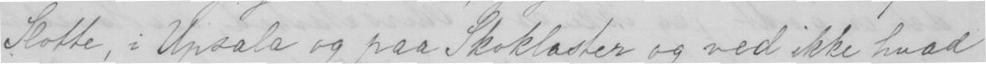Slotte, i Upsala og paa Skoklasten og ved ikke hvad
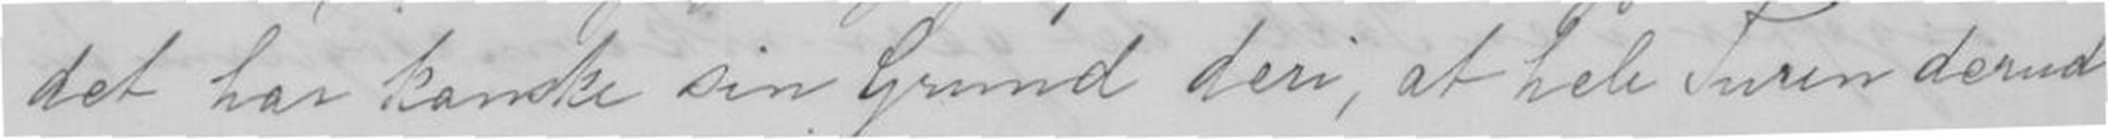det har kanske sin Grund deri, at hele Turen dermed
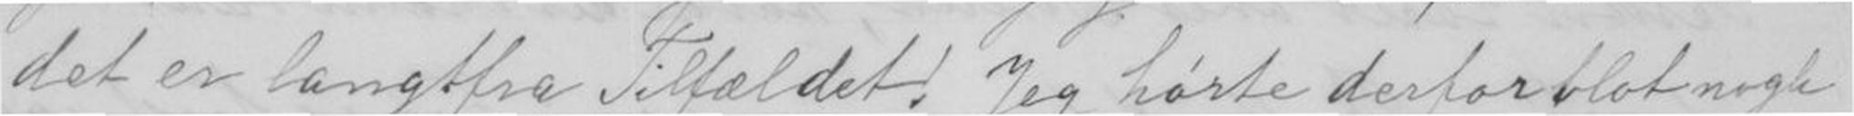det er langtfra Tilfældet! Jeg hørte derfor blot nogle
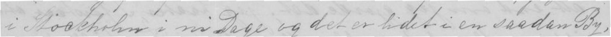i Stockholm i ni Dage og det er lidet i en saadan By,
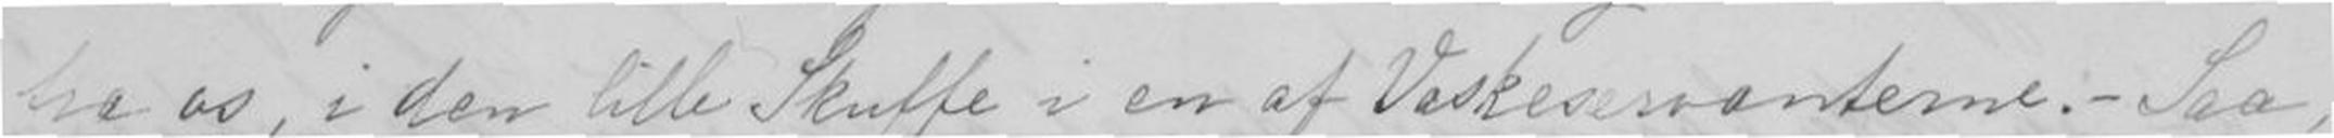fra os, i den lille Skuffen i en af Vaskeservanterne.- saa
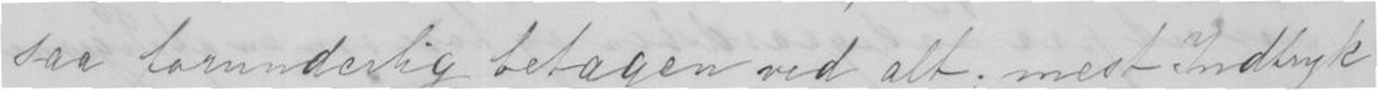saa forunderlig betagen ved alt; mest indtryk
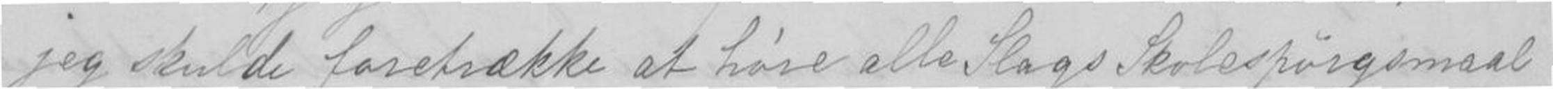jeg skulde foretrække at høre alle Slags Skolespørgsmaal
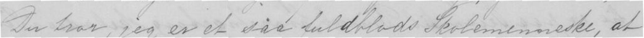Du har, jeg er et saa fuldblods Skolemenneske, at
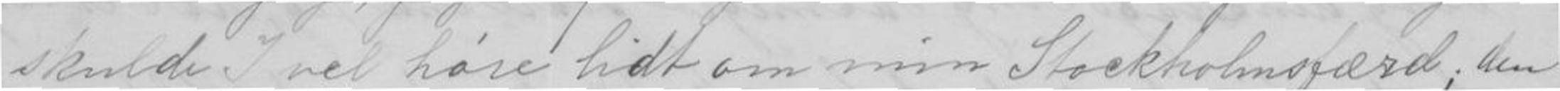skulde vel høre Lidt om min stockholmsfærd, den
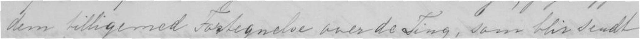dem billige med Fortegnelse over de Ting, som blir sendt
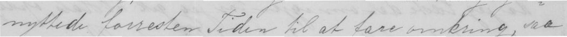nyttede forresten tiden til at fare omkring, saa
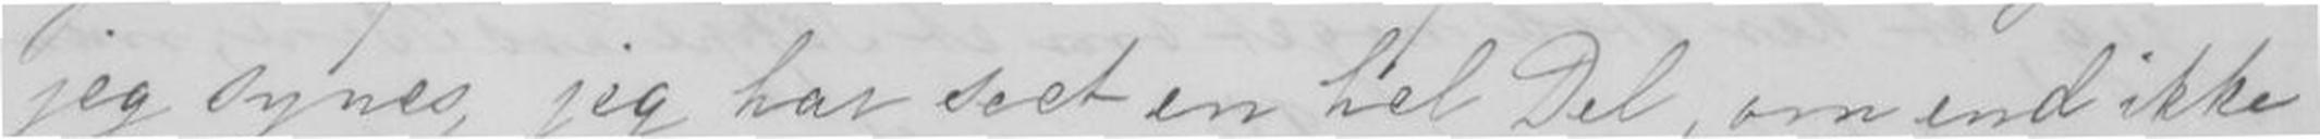jeg øynes, jeg har seet en hel Del, om end ikke
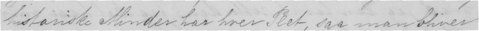historiske Minder har hver Plet, saa man bliver
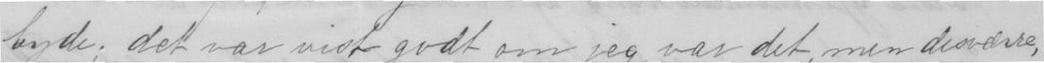byde; det var vist godt om jeg var det, men desverre,
